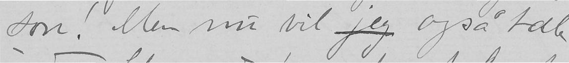son! Men nu vil jeg også tale
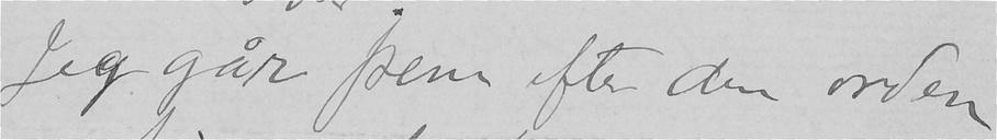Jeg går frem efter den orden
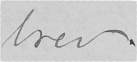brev.
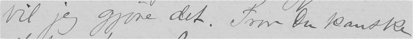vil jeg gjøre det. Tror Du kanske
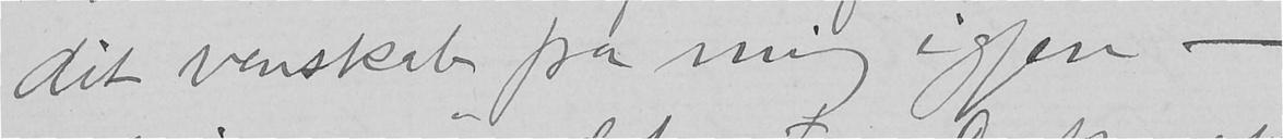dit venskab fra mig igjen -
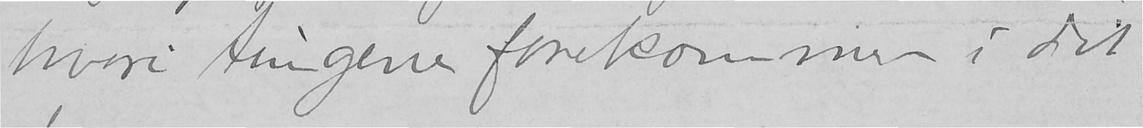hvori tingene forekommer i dit
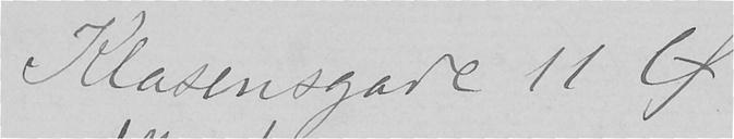Klasensgade 11 Ø
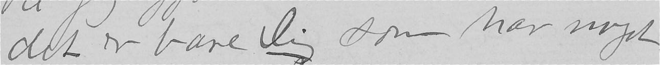det er bare Dig som har noget
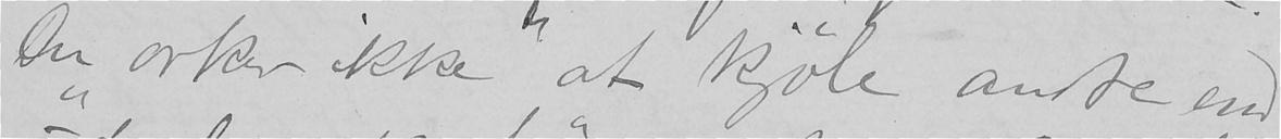Du orker ikke at kjøbe andde end
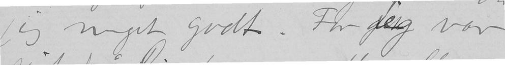jeg meget godt. For jeg var
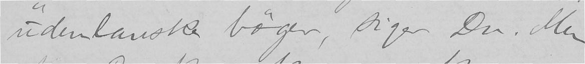udenlanske bøger, siger Du. Men
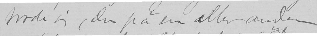trode jeg, Du på en ellr anden
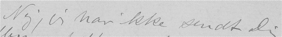Nej, jeg har ikke sendt dig
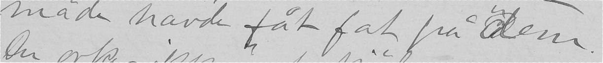måde havde fåt fat på dem.
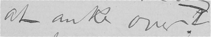at anke over?
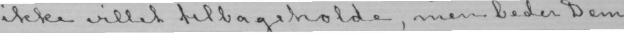ikke villet tilbageholde, men beder Dem
